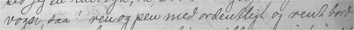vogn, saa! ren og pen med ordentligt og rent bord
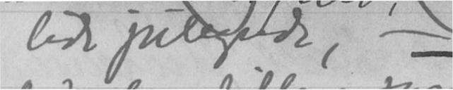lidt julepyndt, -
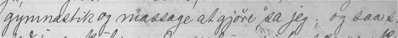gymnastik og massage at gjøre" sa jeg; og saa s
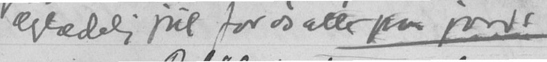glædelig jul for os alle pa jord
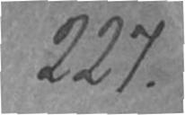227.
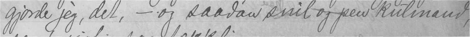gjorde jeg, det, - og saadan snil og pen kulmand,
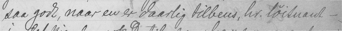saa godt, naar en er daarlig tilbens, hr. løitnant -
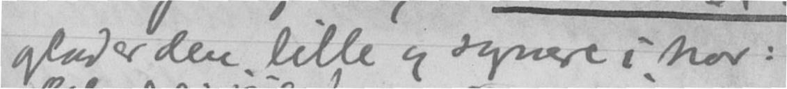glad er den lille o synere i nor:
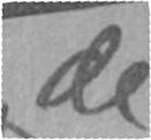de
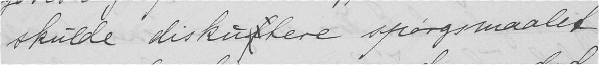skulde diskutere spørgsmaalet
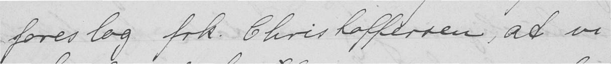foreslog frk. Christoffersen, at vi
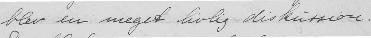blev en meget livlig diskussion.
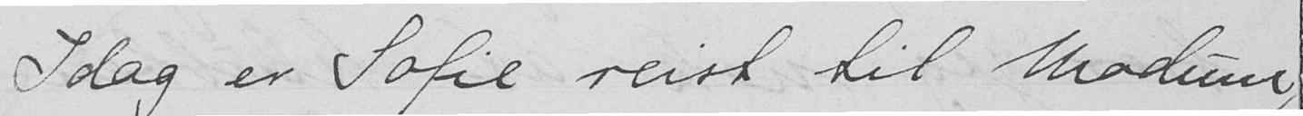Idag er Sofie reist til Modum,
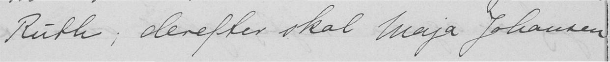Ruth, derefter skal Maja Johansen
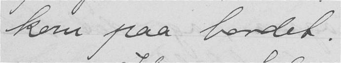kom paa bordet.
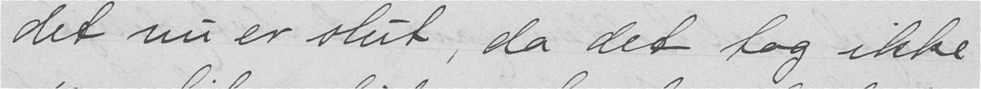det nu er slut, da det tog ikke
